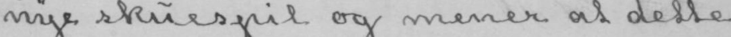nye skuespil og mener at dette
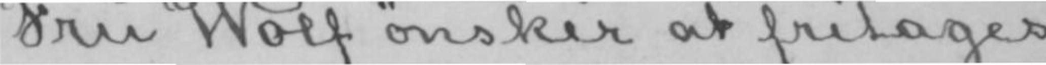Fru Wolf ønsker at fritages
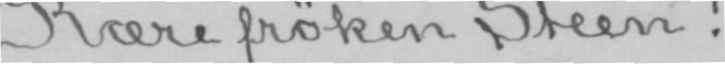Kære frøken Steen!
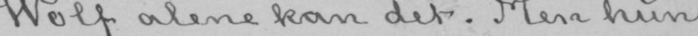Wolf alene kan det. Men hun
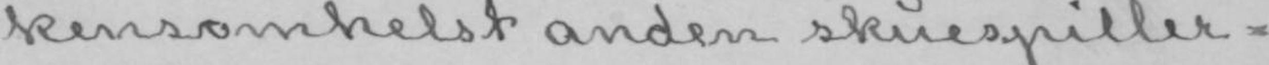kensomhelst anden skuespiller-
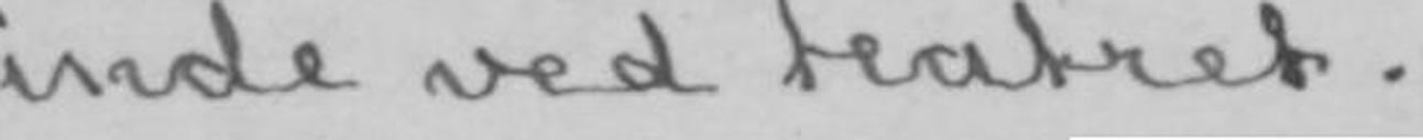inde ved teatret.
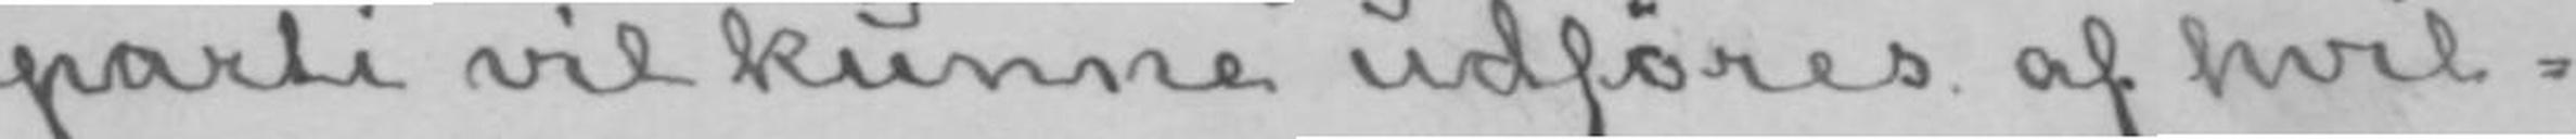parti vil kunne udføres af hvil-
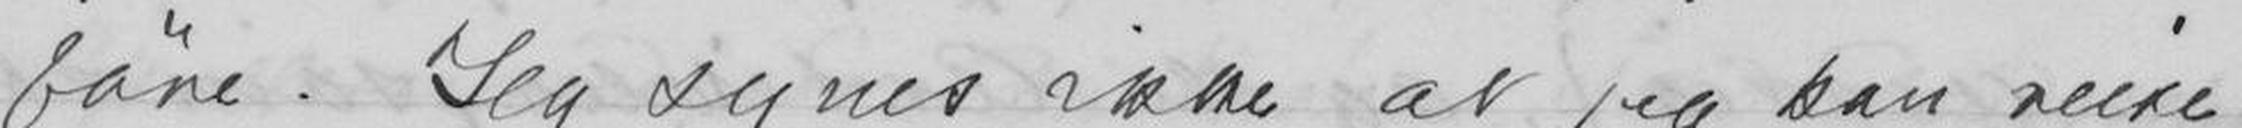føre. Jeg synes ikkeat jeg kan reise
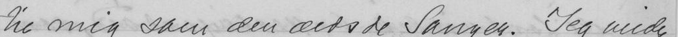til mig som den ældsde Sangen. Jeg vilde
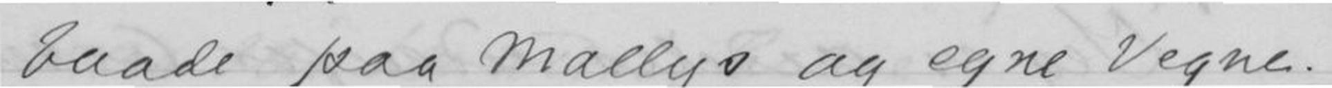baade paa Mallys og egne Vegne.
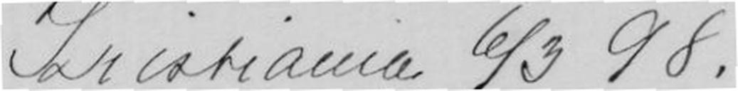Kristiania 6/3 98
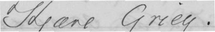Kjære Grieg
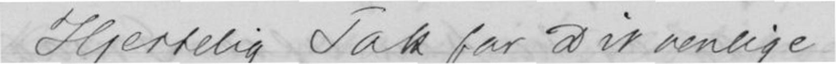Hjertelig Tak for Din venlige
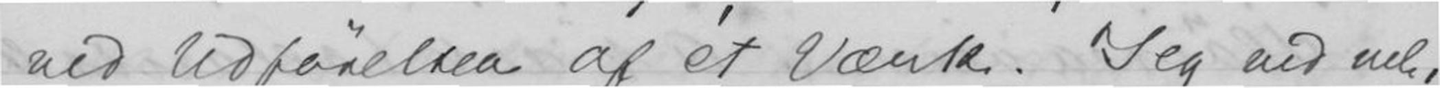ved Udførelsen af et Værk. Jeg ved
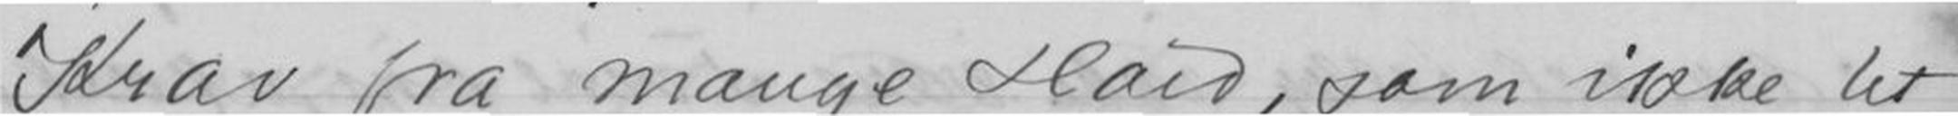Krav fra mange Hold, som ikke let
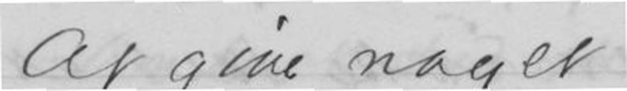At give noget
